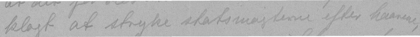klogt at stryke statsmagterne efter haarene,
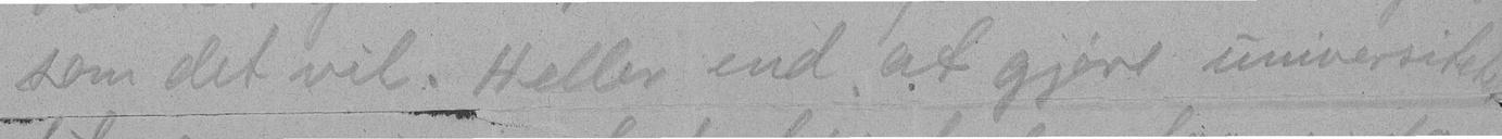som det vil. Heller end at gjøre univesitetet
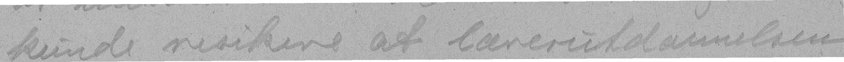kunde risikere at lærerutdannelsen
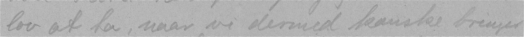lov at ta, naar vi dermed kanske bringer
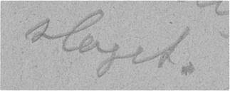slaget.
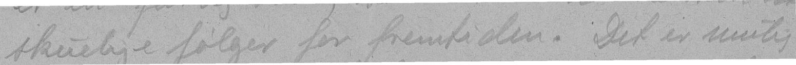skuelige følger for fremtiden. Det er mulig
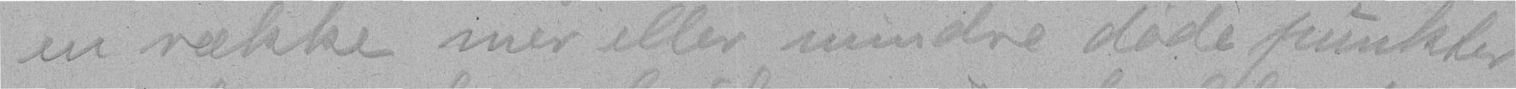en række mer eller mindre døde punkter
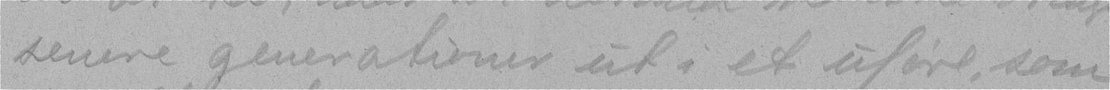senere generationer ut i et uføre som
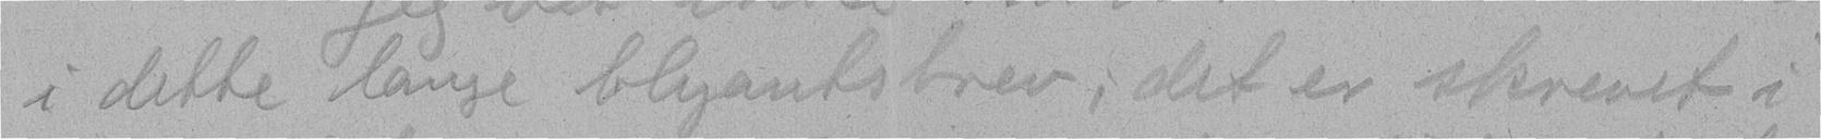i dette lange blyantsbrev; det er skrevet i
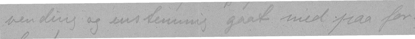vending og enstemmig gaat med paa for-
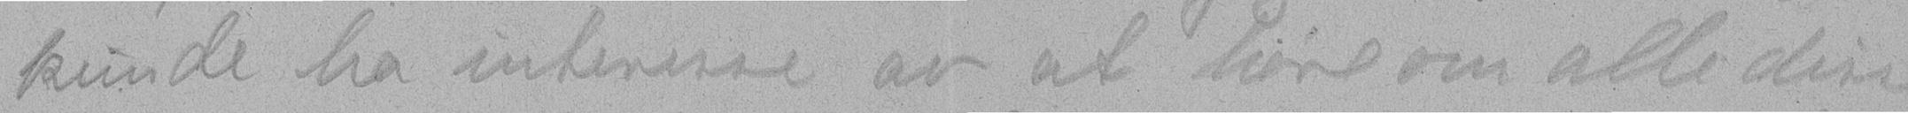kunde ha interesse av at høre om alle disse
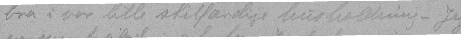bra i vor lille stilfærdige husholdning - Jeg
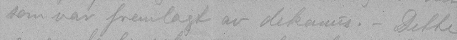som var fremlagt av dekanus: - Dette
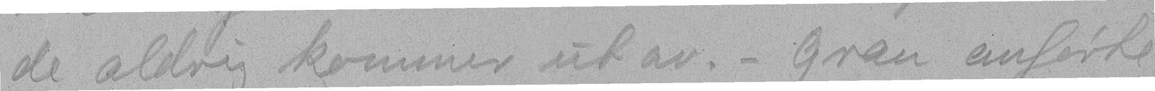de aldrig kommer ut av. - Gran anførte
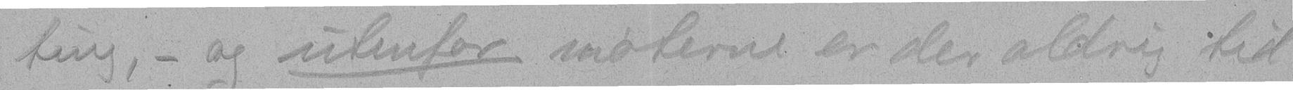ting,- og utenfor møterne er der aldrig tid
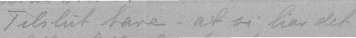Tilslut bare - at vi har det svært
